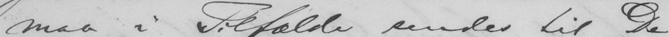maa i Tilfælde sendes til De-
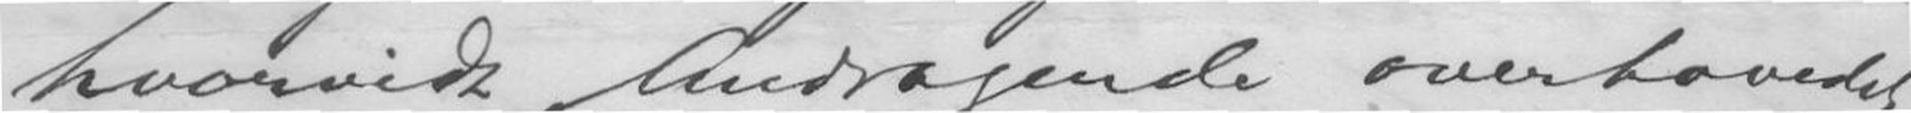hvorvidt Andragende overhovedet
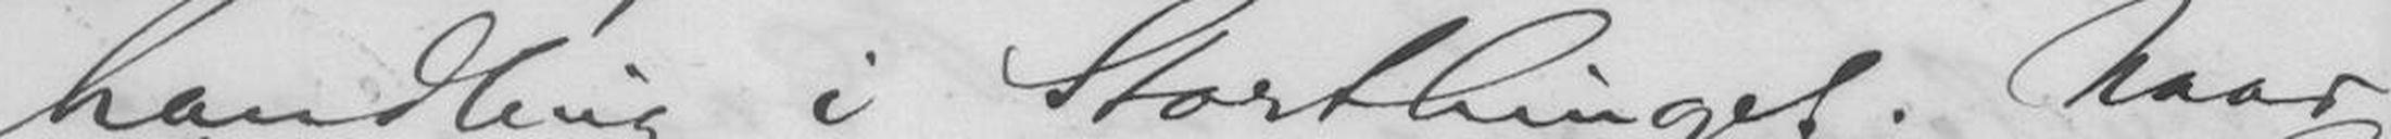handling i Storthinget. Naar
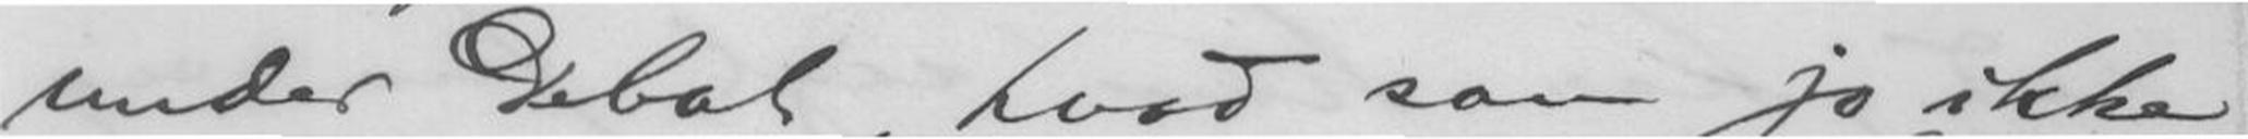under Debat, hvad som jo ikke
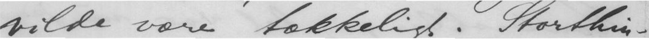vilde være tækkeligt. Storthin-
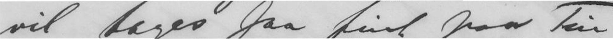vil tages saa fint paa Tin-
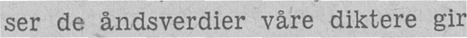ser de åndsverdier våre diktere gir
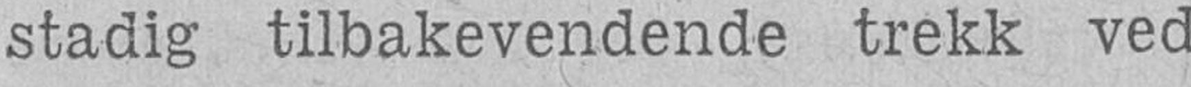stadig tilbakevendende trekk ved
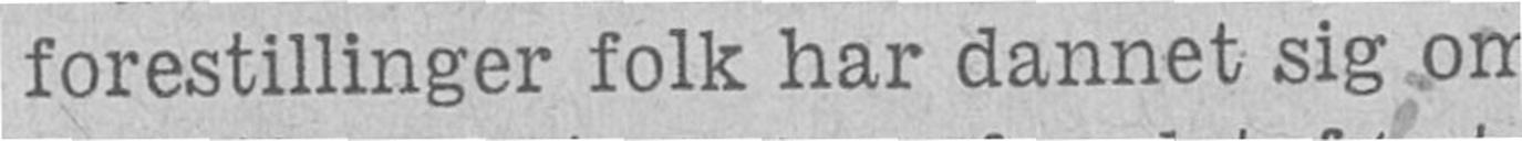forestillinger folk har dannet sig om
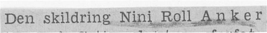Den skildring Nini Roll Anker
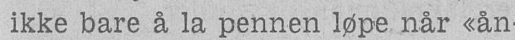ikke bare å la pennen løpe når "ån
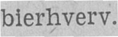bierhverv
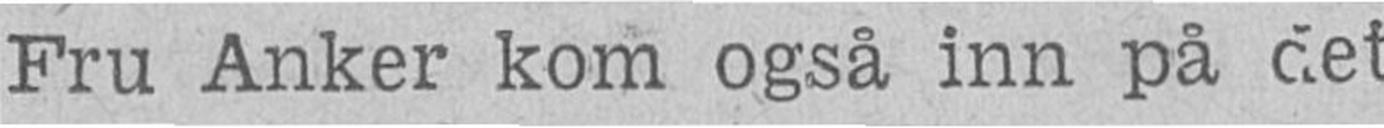Fru Anker kom også inn på det
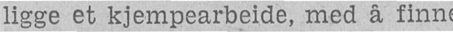ligge et kjempearbeide, med å finne
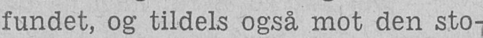fundet, og tildels også mot den sto-
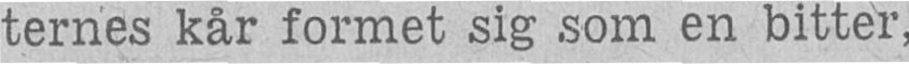ternes kår formet sig som en bitter,
